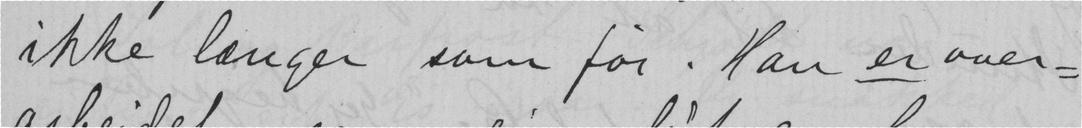ikke længer som før. Han er over-
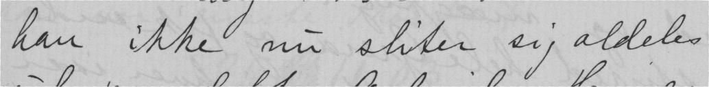han ikke nu sliter sig aldeles
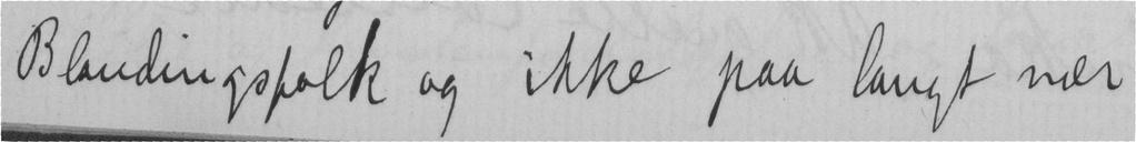Blandingsfolk og ikke paa langt nær
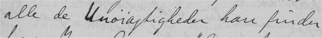alle de unøiagtigheder han finder
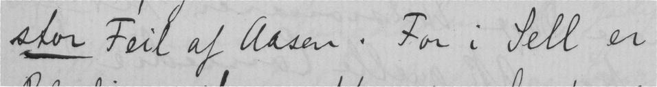stor Feil af Aasen. For i Sell er
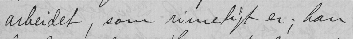arbeidet, som rimeligt er; han
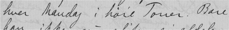hver mandag i høie Toner. Bare
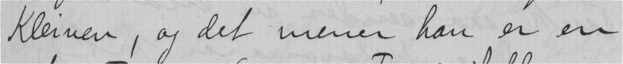Kleiven, og det mener han er en
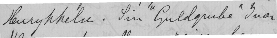Henrykkelse. Sin "Guldgrube" Ivar
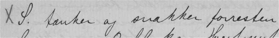x I. tænker og snakker forresten
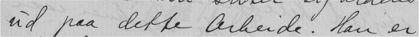ud paa dette arbeide. Han er
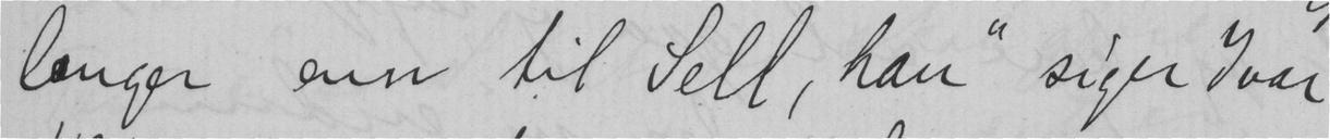længer enn til Sell, han" siger Ivar
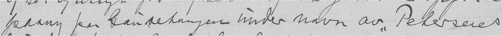paany paa Gausetangen under navn av "Petersens
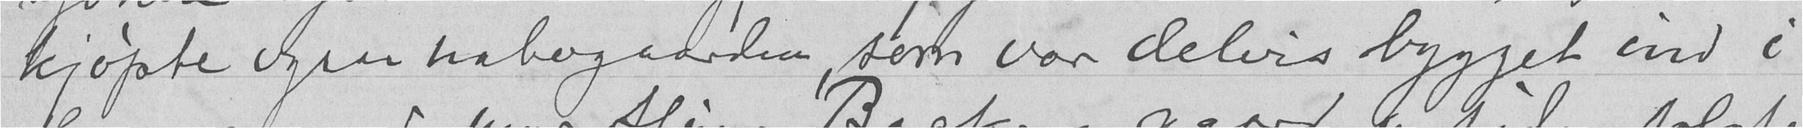kjøpte ogsaa nabogaarden, som var delvis bygget ind i
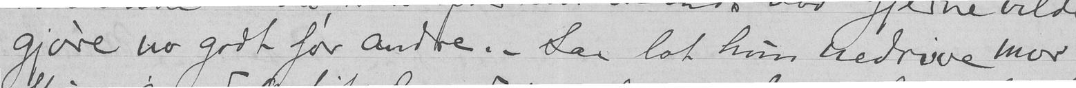gjøre no godt for andre. - Saa lot hun nedrive mor
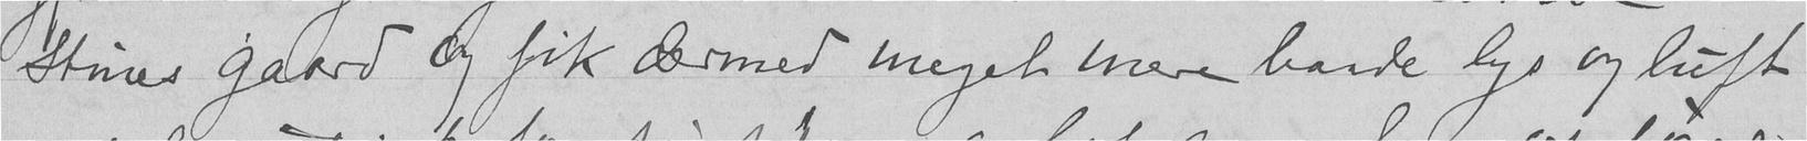Stines gaard og fik dermed meget mere baade lys og luft
